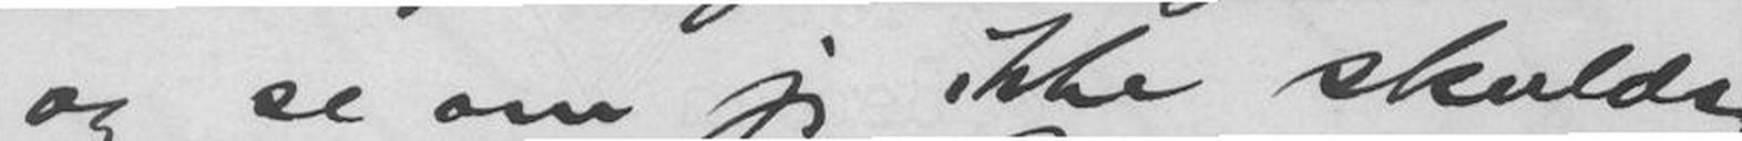og se om jeg ikke skulde
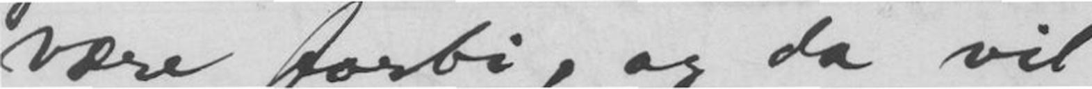være forbi, og da vil
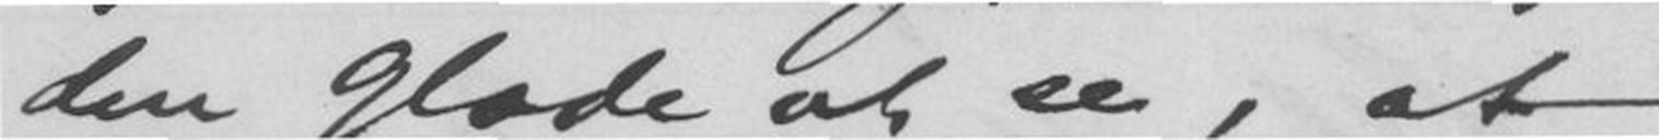den Glæde at se, at
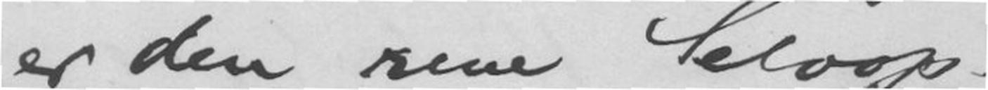er den rene Selvop-
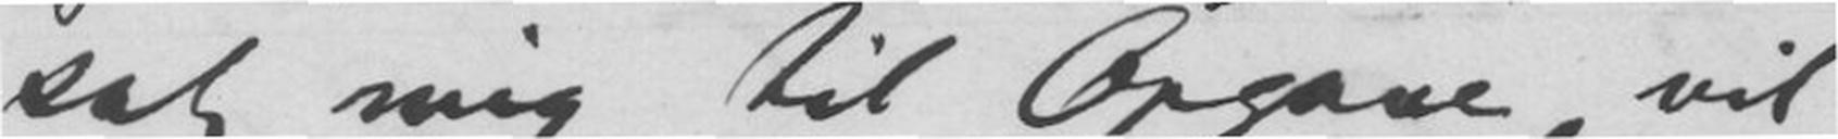sat mig til Opgave, vil
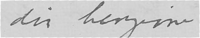din hengivne
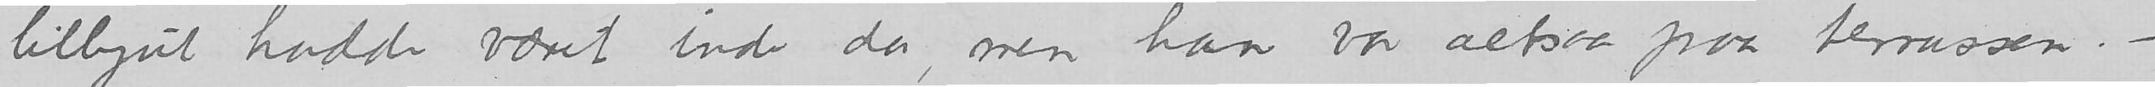lillegut hadde været inde da, men han var altsaa paa terrassen. –
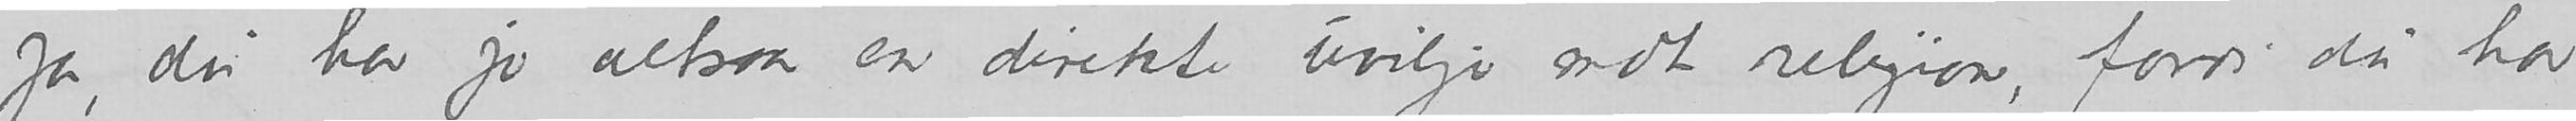Ja, du har jo altsaa en direkte uvilje mot religion, fordi du har
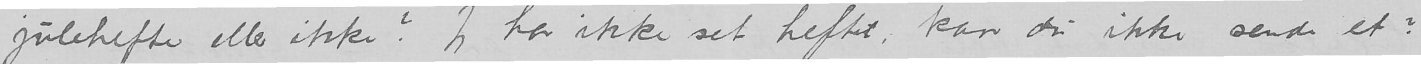julehefte eller ikke? Jeg har ikke sett heftet, kan du ikke sende et?
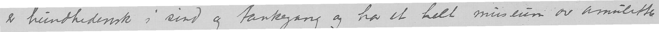er hundhedensk i sind og tankegang og har et helt museum av amuletter
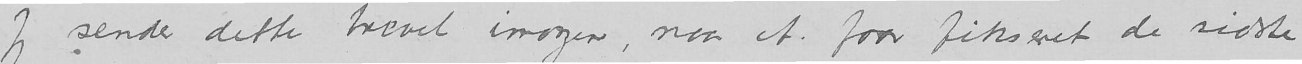Jeg sender dette brevet imorgen, naar A. faar fikseret de sidste
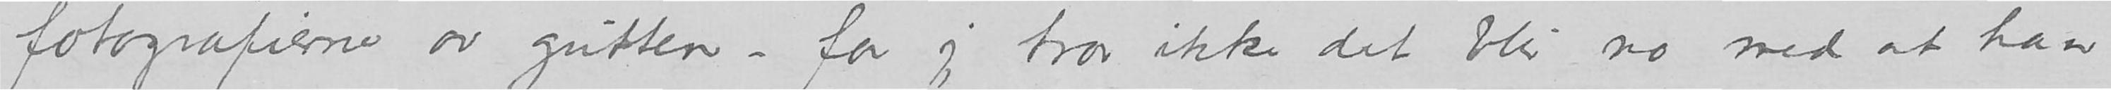fotografierne av gutten – for jeg tror ikke det blir no med at han
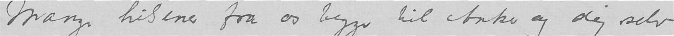Mange hilsener fra os begge til Anker og dig selv
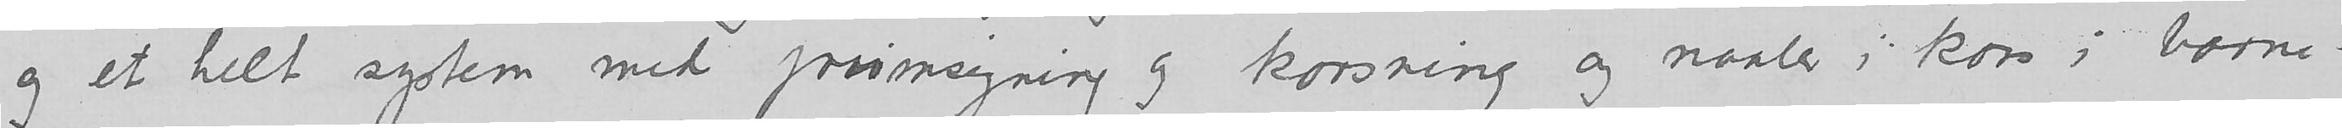og et helt system med primsigning og korsring og naaler i kors i barne-
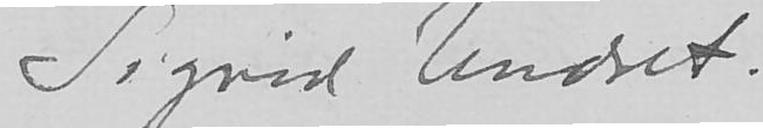Sigrid Undset.
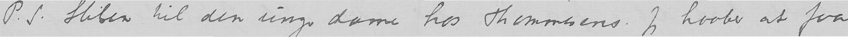P.S. Hilsen til den unge dame hos Thommesens. Jeg haaber at faa
